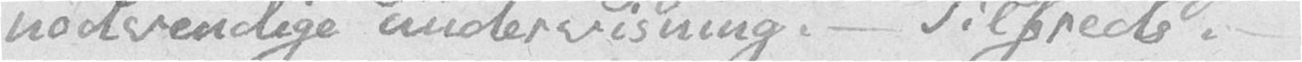nødvendige undervisning. – Tilfreds. –
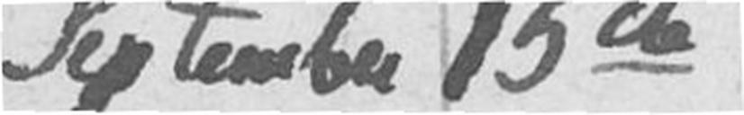September 15de
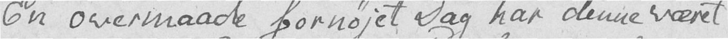En overmaade fornøjet Dag har denne været
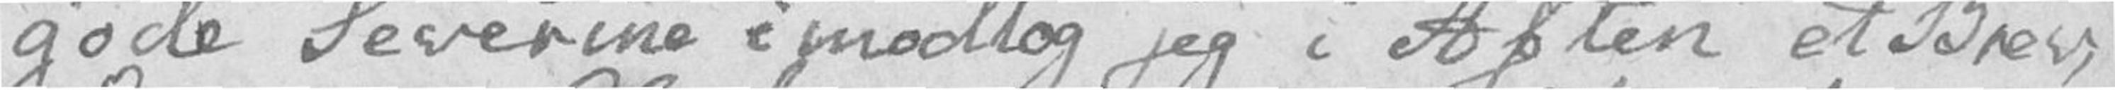gode Severine imodtog jeg i Aften et Brev,
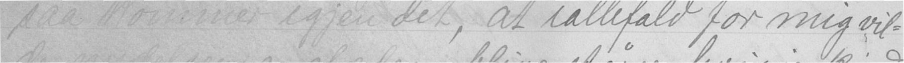saa kommer igjen det, at iallefald for mig vil-
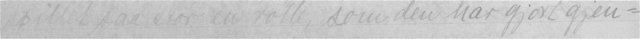spillet saa stor en rolle, som den har gjort gjen-
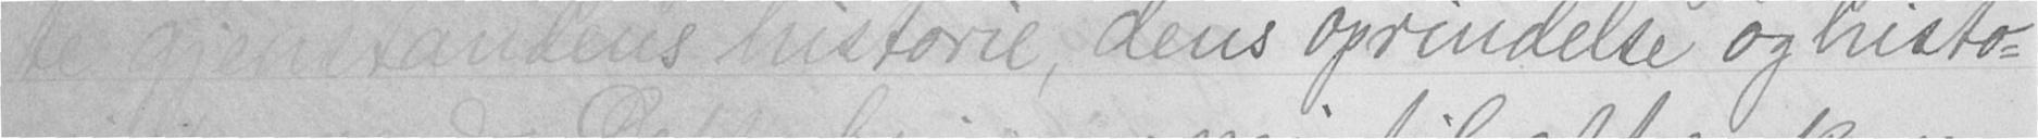te gjenstandens historie, dens oprindelse og histo-
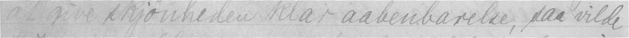at give skjønheden klar aabenbarelse, saa vilde
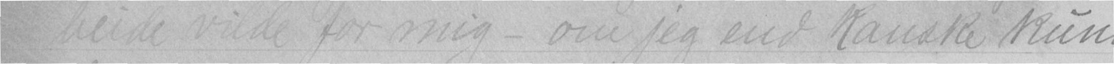heide vilde for mig - om jeg end kanske kunde
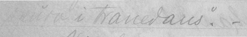spurv i tranedans". -
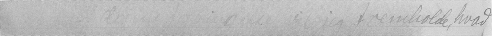vil jeg fremholde, hvad
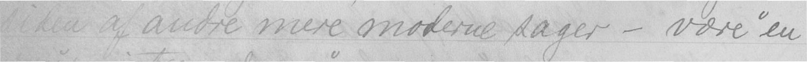siden af andre mere moderne sager - være "en
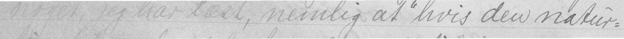noget, jeg har læst, nemlig, at "hvis den natur-
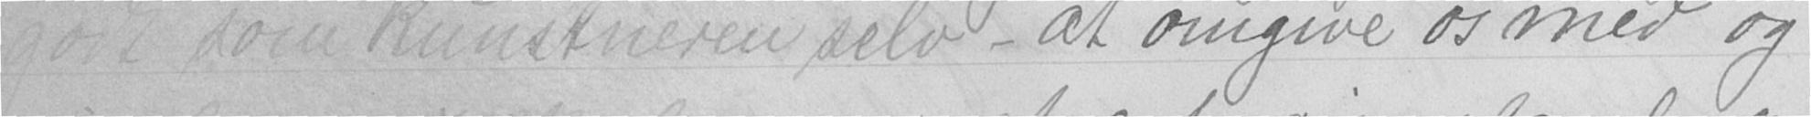godt som kunstneren selv - at omgive os med og
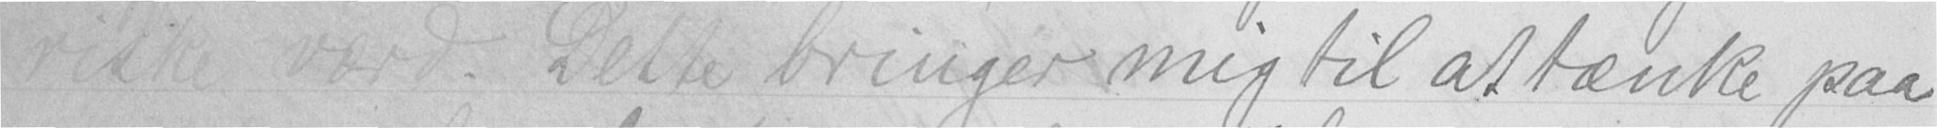riske værd. Dette bringer mig til at tænke paa
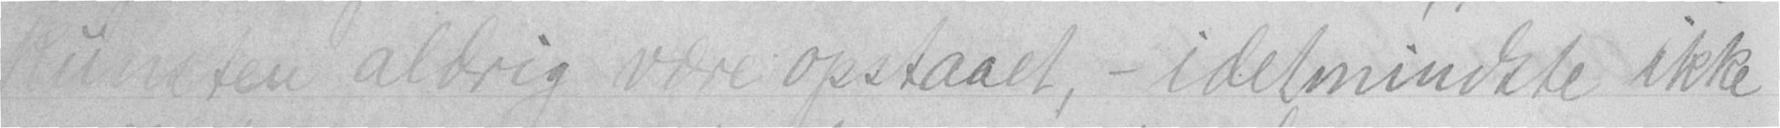kunsten aldrig være opstaaet, - i det mindste ikke
